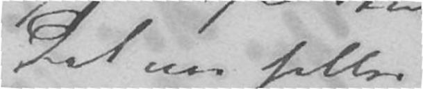Det er heller
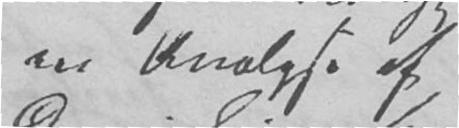en Analyse af
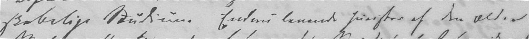skabelige Studium. Endnu levende Jurister af den ældre
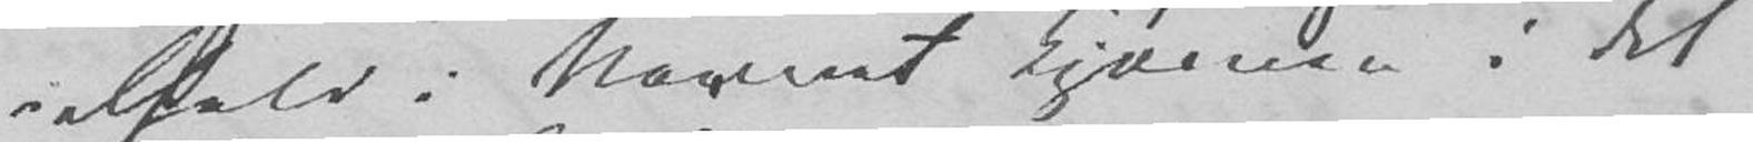ialfald i Navnet Kjærnen i det
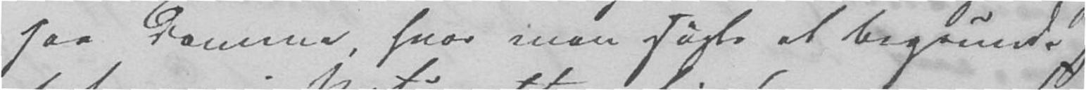saa Dommer, hvor man søgte at begrunde
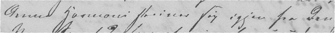denne Harmoni skriver sig igjen fra den
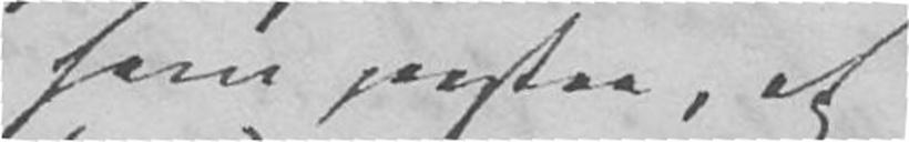ham paastaa, at
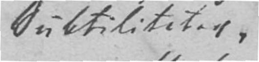Subtiliteter,
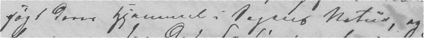søgt deres Hjemmel i Sagens Natur, og
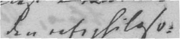den retsphiloso-
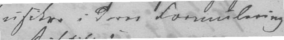usikre i deres Formulering
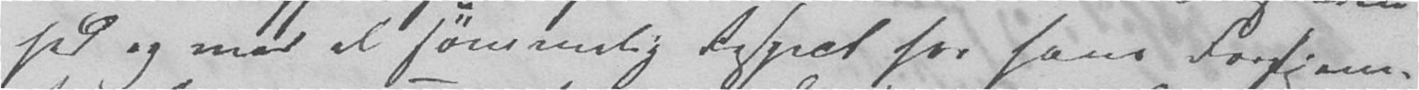hed og med al sømmelig Respect for hans Fortjen-
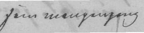som mangengang
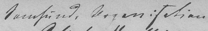Samfunds Organisation.
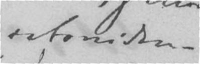retsviden-
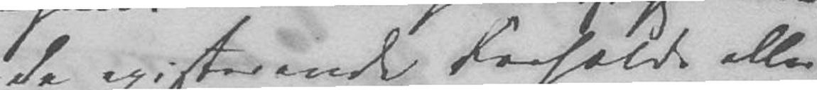de existerende Forholde eller
